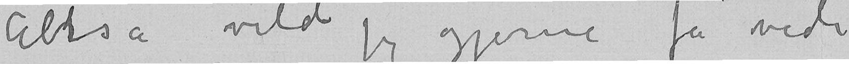Altså vilde jeg gjerne få vide
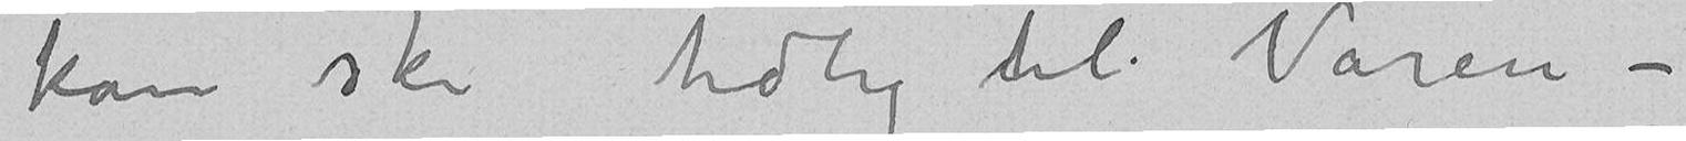kan ske tidlig til Våren –
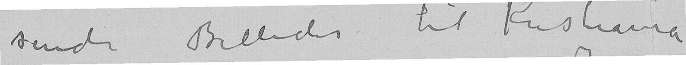sende Billeder til Kristiania
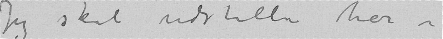Jeg skal udstille her i
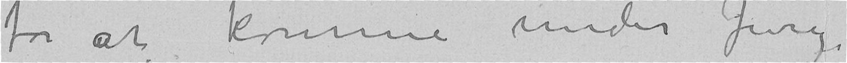for at komme under Jury.
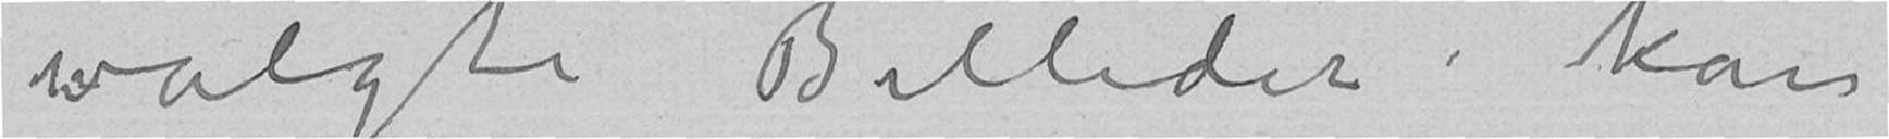valgte Billeder kan
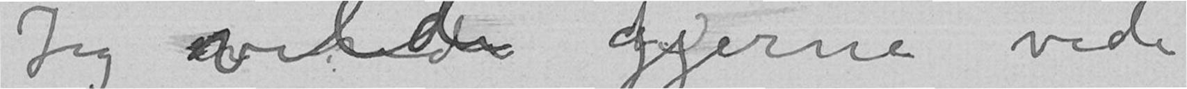Jeg vilde gjerne vide
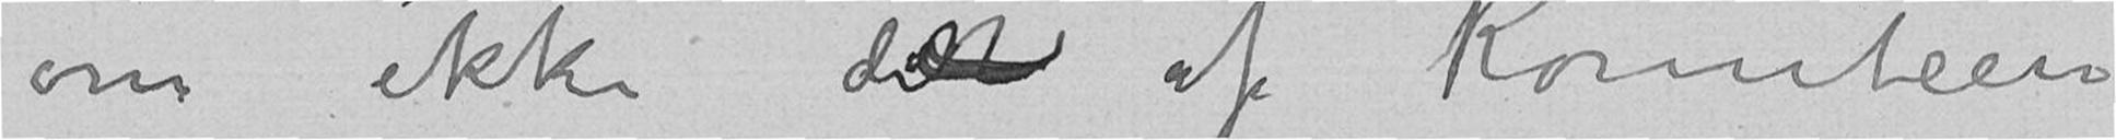om ikke de af Komiteen
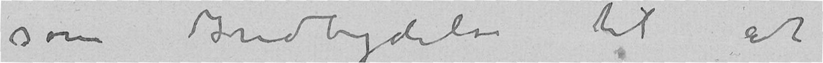som Indbydelse til at
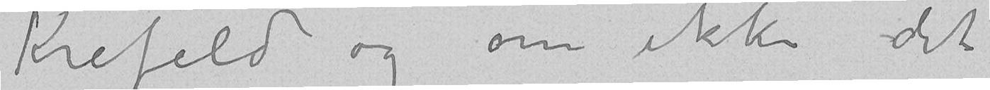Krefeld og om ikke det
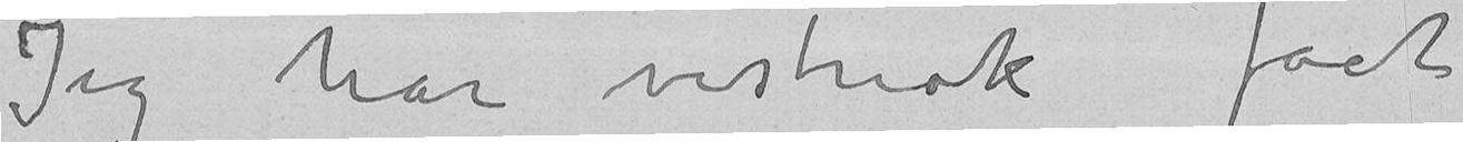Jeg har vistnok fået
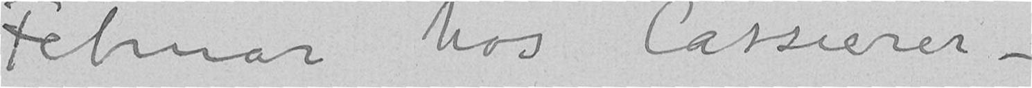Februar hos Cassirer –
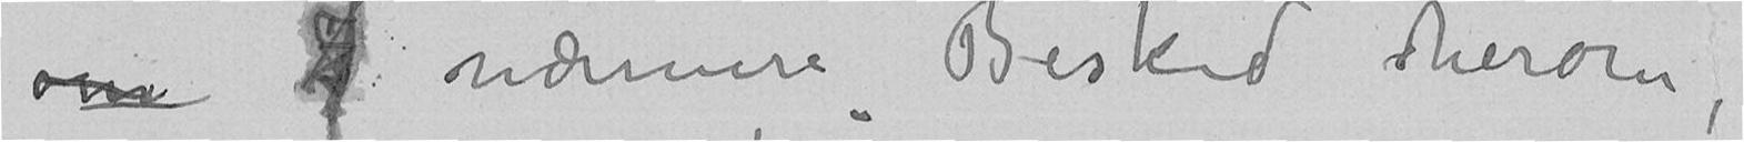om nærmere Besked herom,
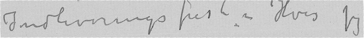Indleveringsfrist – Hvis jeg
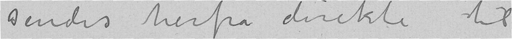sendes herfra direkte til
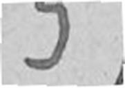5)
